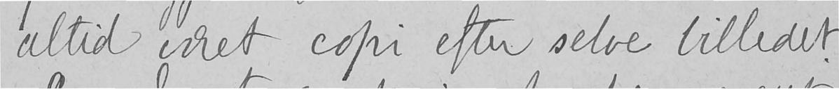altid været copi efter selve billedet.
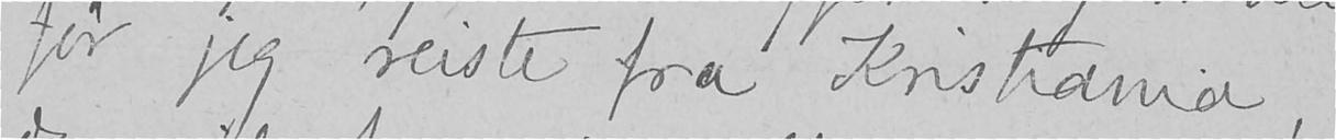før jeg reiste fra Kristiania,
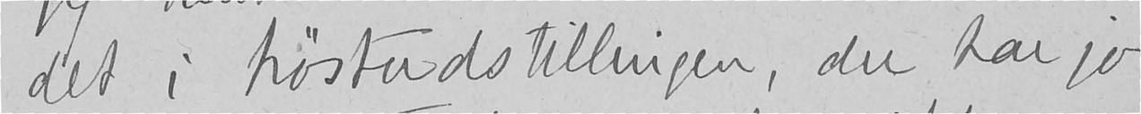det i høstudstillingen, du har jo
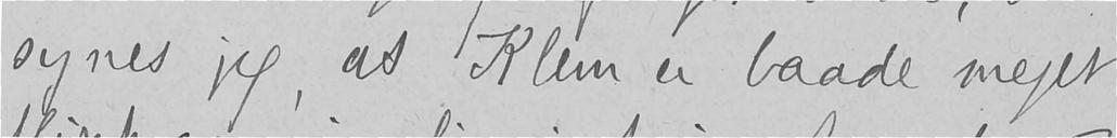synes jeg, at Klem er baade meget
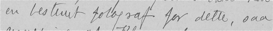en bestemt fotograf for dette, saa
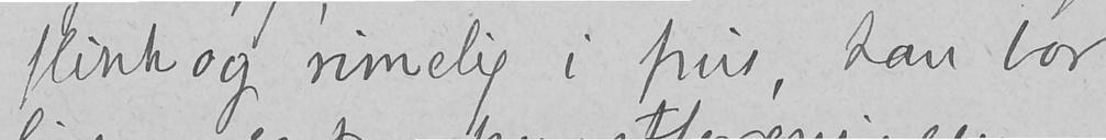flink og rimelig i pris, han bor
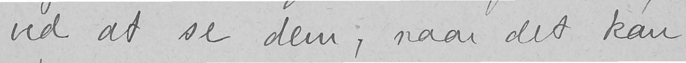ved at se dem, naar det kan
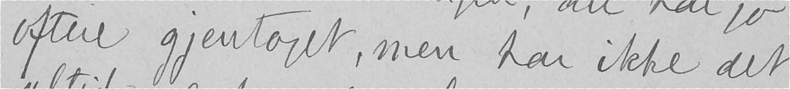oftere gjentaget, men har ikke det
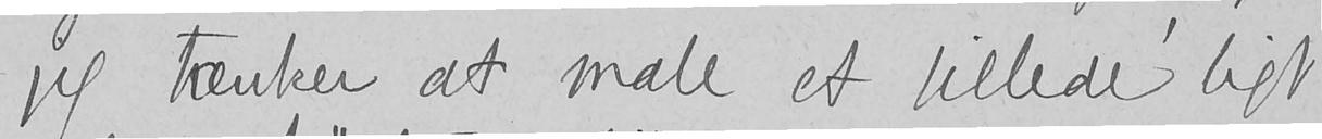jeg tænker at male et billede ligt,
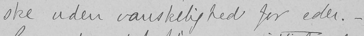ske uden vanskelighed for eder. -
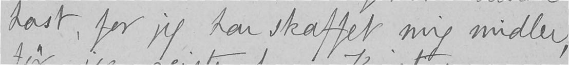hast, for jeg har skaffet mig midler,
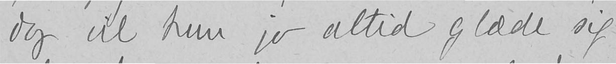dog vil hun jo altid glæde sig
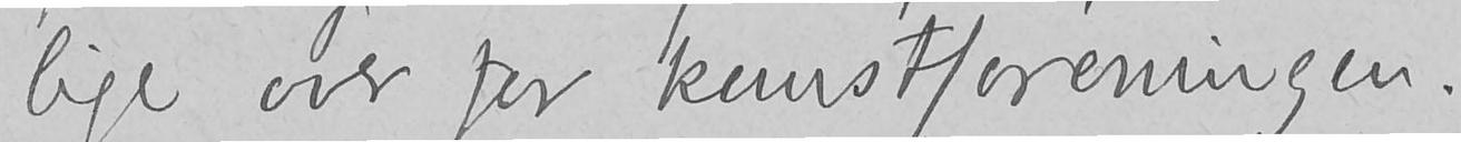lige over for kunstforeningen.
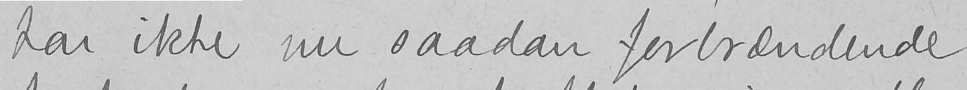har ikke nu saadan forbrændende
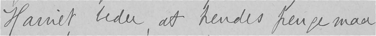Harriet beder at hendes penge maa
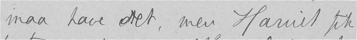maa have det, men Harriet fik
